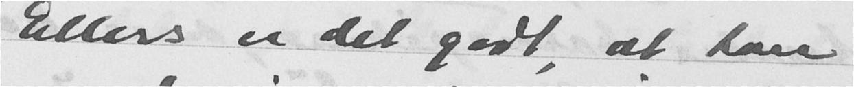Ellers er det godt, at han
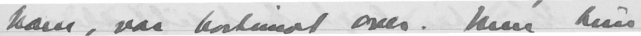ham, var bortimot over. Men hun
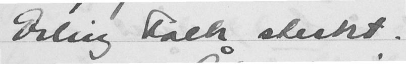Erling Falk sterkt.
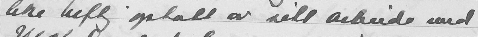like heftig optatt av sitt arbeide med
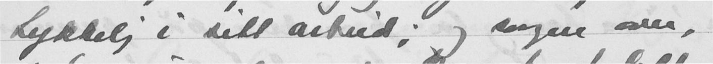Lykkelig i sitt arbeid; og sorgen over,
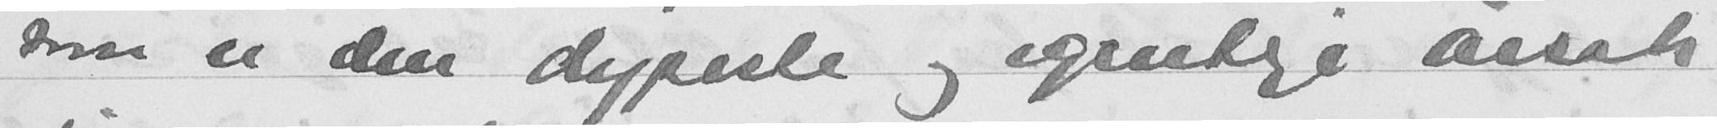som er den dypeste og egentlige årsak
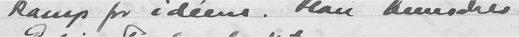kamp for ideen. Han beundrer
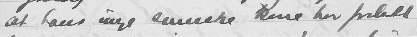at hans unge svenske kone har forlatt
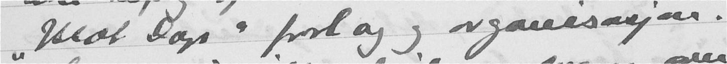"Mot Sops" foretag og organisasjonen.
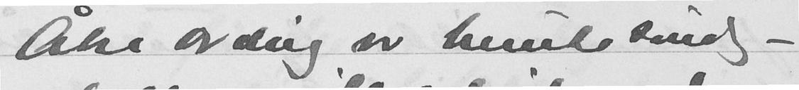Åke av deig er hentebandig -
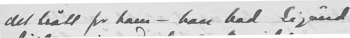det trått for ham - han bad Sigurd
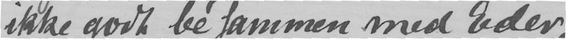ikke godt be sammen med Eder,
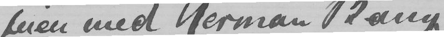men med Herman Bang,
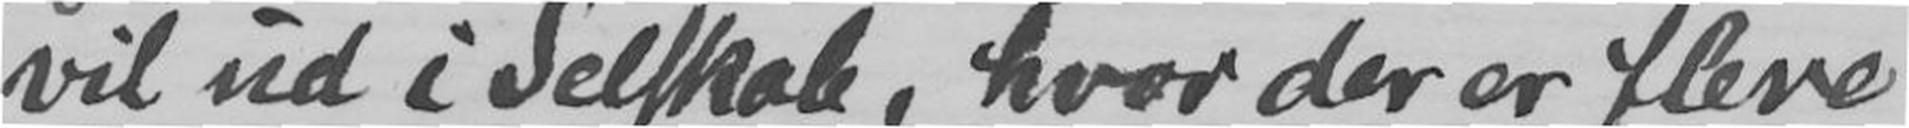vil ud i Selskab, hvor der er flere
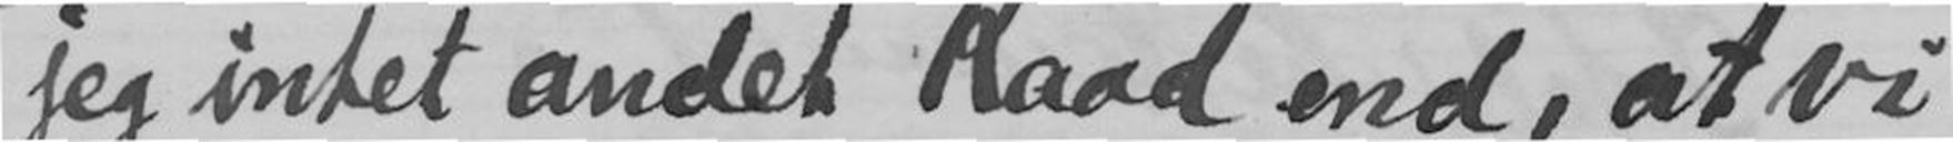jeg intet andet Raad end, at vi
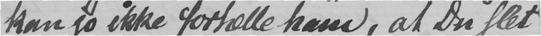kan jo ikke fortælle ham, at Du slet
